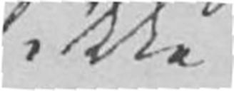ikke
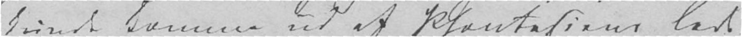kunde komme ud af Phantasiens Lede-
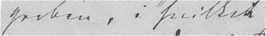greben, i hvilken
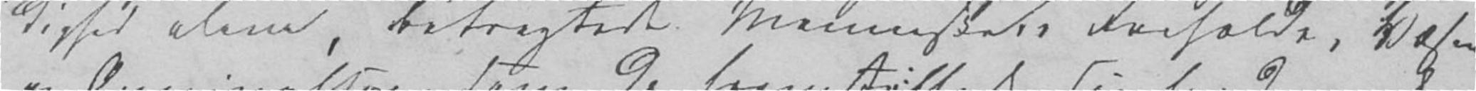dighed alene, betragtede Mennesketes Forholde, Væsen
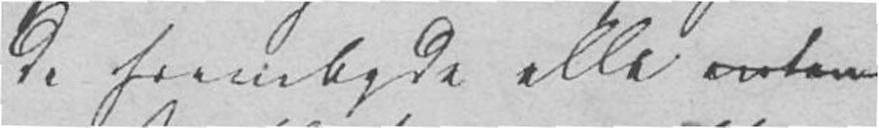de frembyde alle
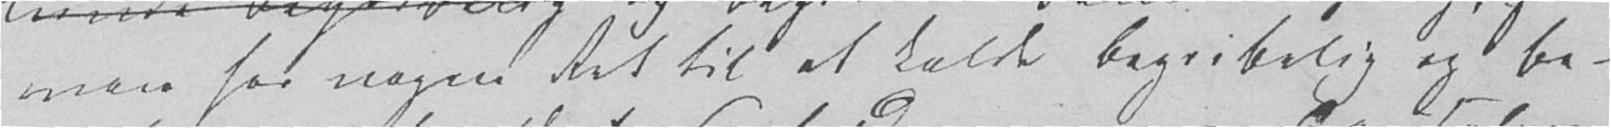man har nogen Ret til at kalde begribelig og be-
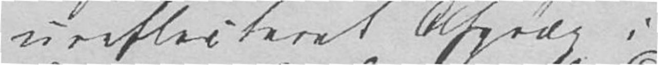ureflecteret Afpræg i
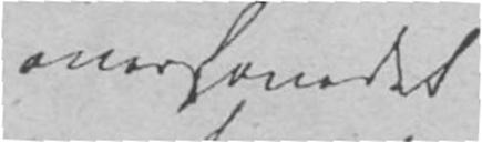overhovedet
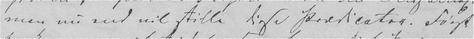man nu end vil stille disse Prædicater. Først
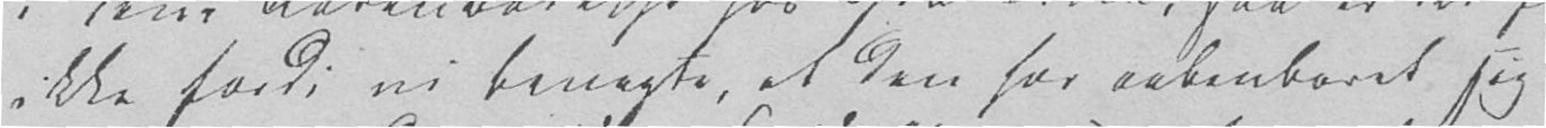ikke fordi vi benægte, at den har aabenbaret sig
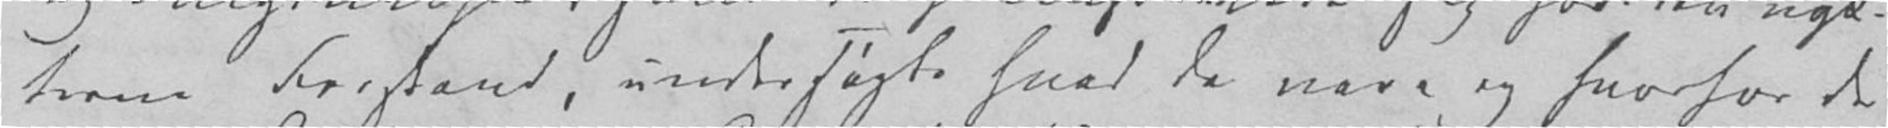terne Forstand, undersøgte hvad de vare og hvorfor de
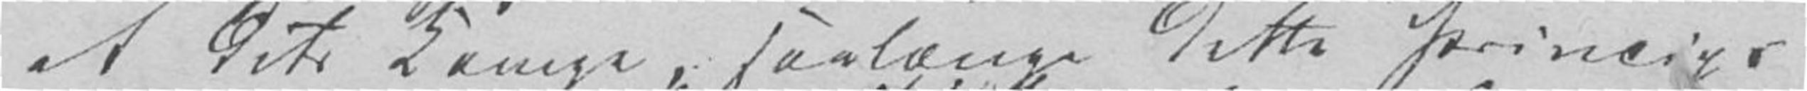at dets Konge, saalænge dette Princips
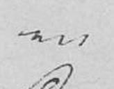en
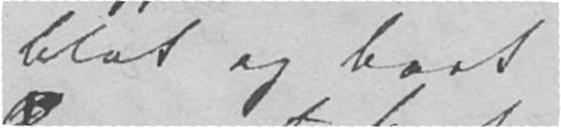blot og bart
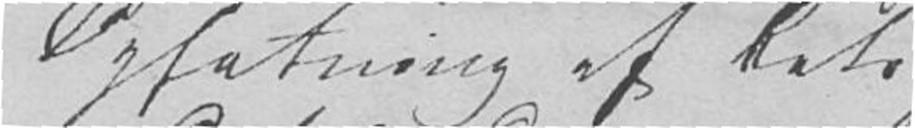Opfatning af Rets
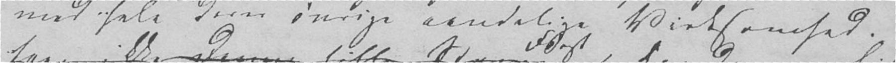med hele deres øvrige aandelige Virksomhed.
